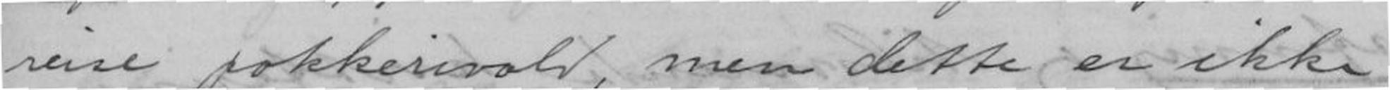reise pokkerivold, men dette er ikke
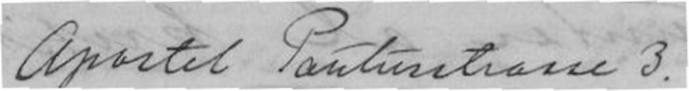Apostel Paulusstrasse 3.
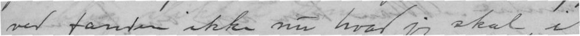ved fanden ikke nu hvad jeg skal, i
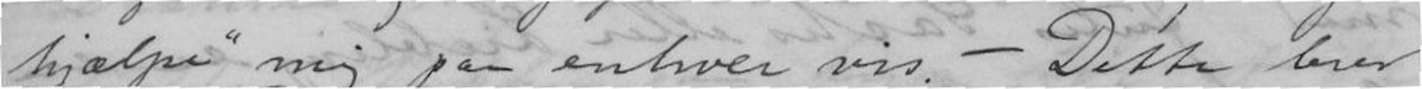"hjælpe" mig paa enhver vis. - Dette brev
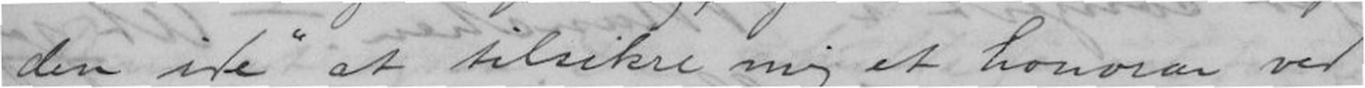den "ide" at tilsikre mig et honorar ved
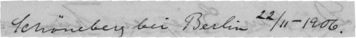Schöneberg dei Berlin 22/11-1906
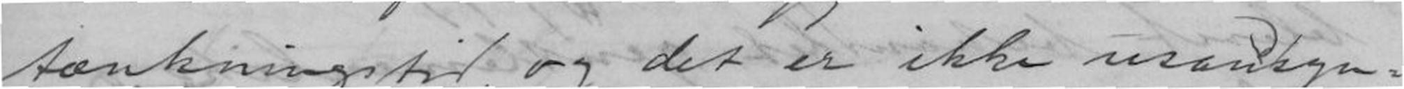tænkningstid, og det er ikke usandsyn-
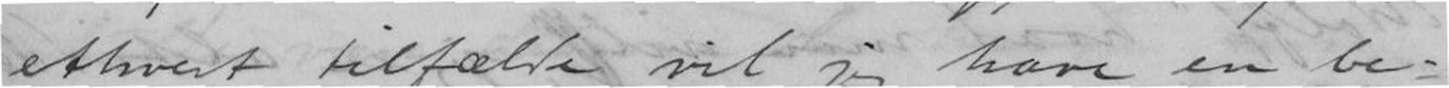ethvert tilfælde vil jeg have en be-
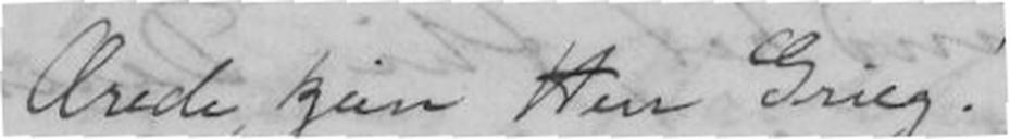Ærede, kjære Herr Grieg!
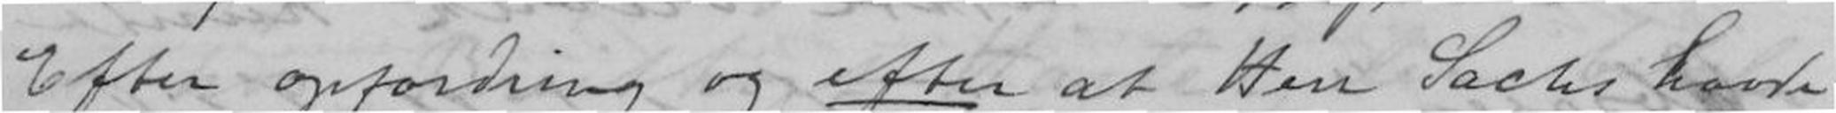Efter opfordring og efter at Herr Sachs havde
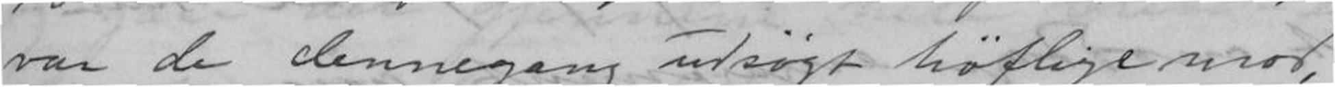var de dennegang udsøgt høflige mod,
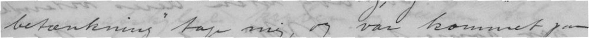betænkning" tage mig, og var kommet paa
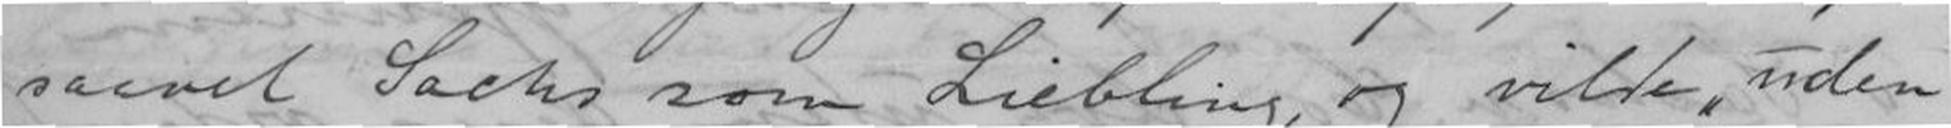saavel Sachs som Liebling, og vilde "uden
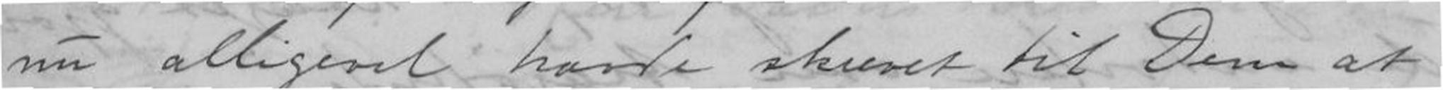u alligevel havde skrevet til Dem at
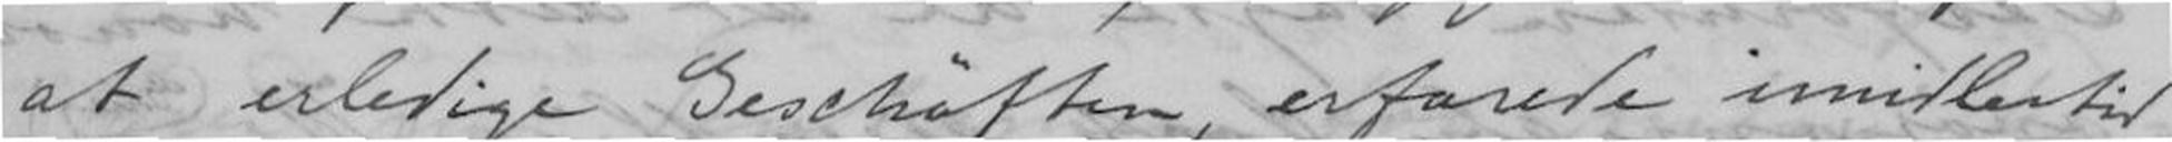at erledige Geschäften, erfarede imidlertid
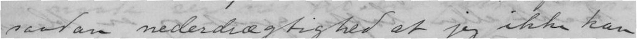saadan nederdrægtighed at jeg ikke kan
